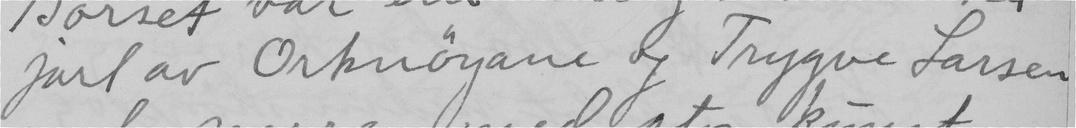jarl av Orknöyane og Trygve Larsen
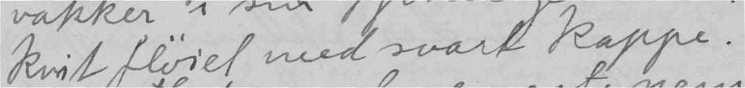kvit flöiel med svart kappe.
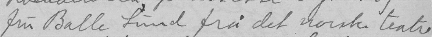fru Balle Lund frå det norske Teatre
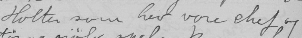Holter som hev vore chef og
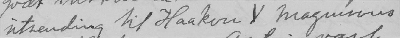utsending til Haakon V Magnussons
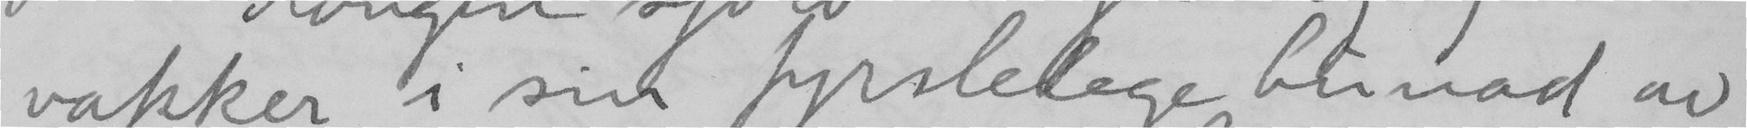vakker i sin fyrstelege bunad av
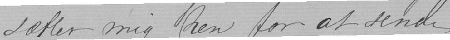sætter mig hen for at sende
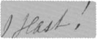I Hast!
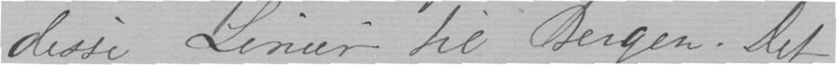disse Linier til Bergen. Det
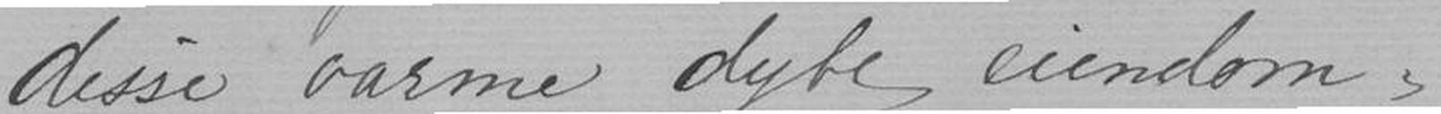disse varme dype eiendom-
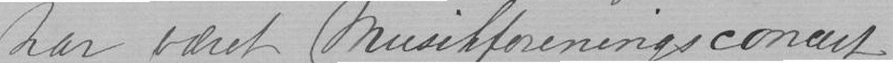har været Musikforeningsconcert
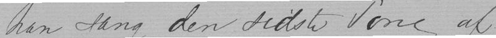han sang den sidste Tone af
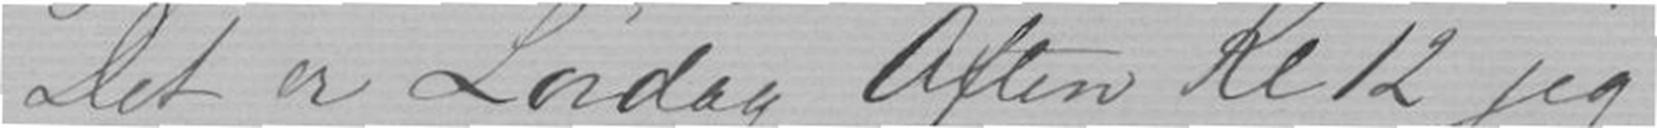Det er lørdag Aften Kl 12 jeg
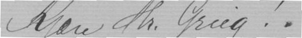Kjære Hr. Grieg!
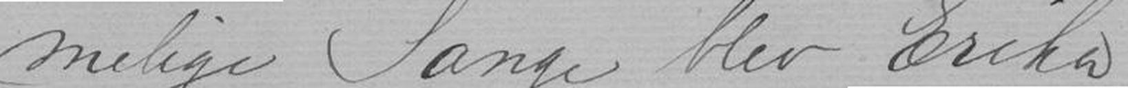melige Sange blev Erika
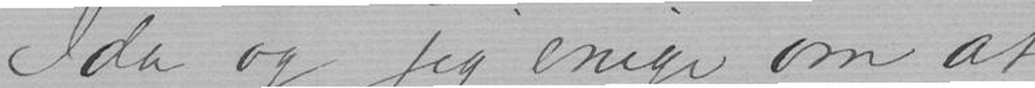Ida og jeg enige om at
